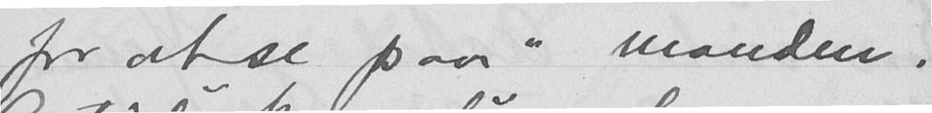for at "se paa" manden.
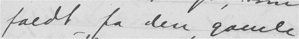faldt fra den gamle
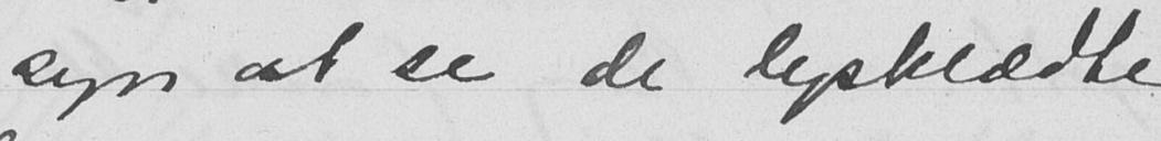syn at se de lysklædte
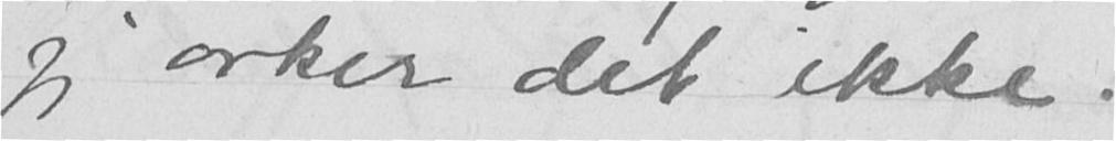jeg orker det ikke.
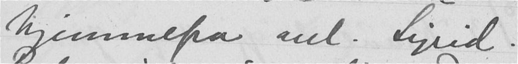hjemmefra anl. Sigrid.
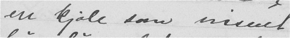en kjole som vissent
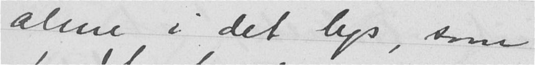alene i det lys, som
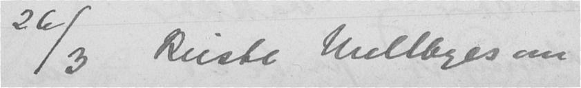26/3 Reiste Mellbyes om
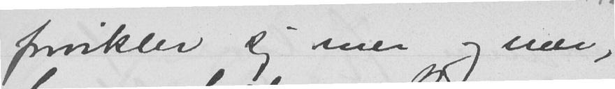forvikler sig mer og mer,
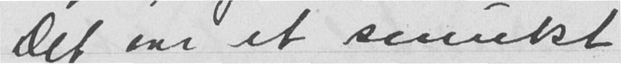Det var et smukt
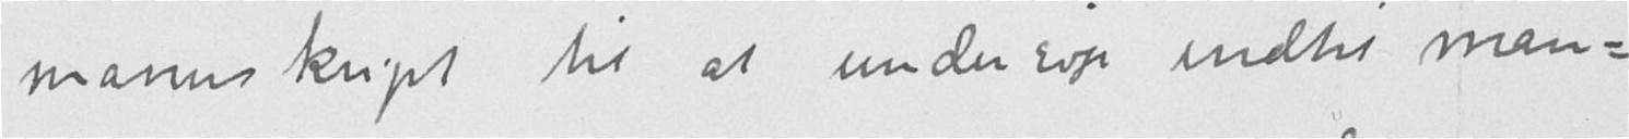manuskript til at undersøge indtil man-
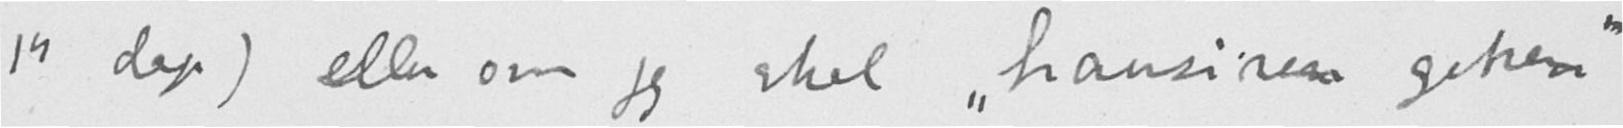14 dage) eller om jeg skal "hausiren gehen"
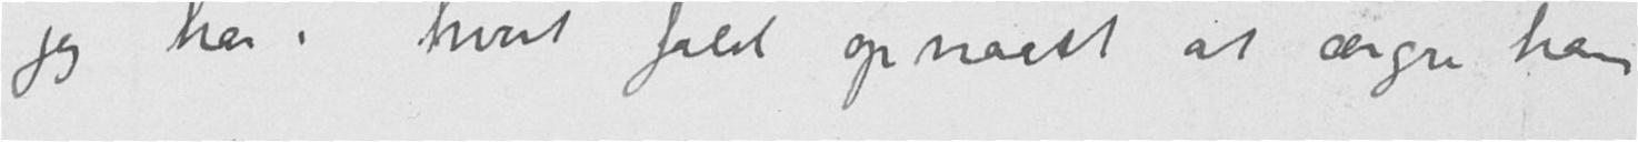jeg har i hvert fald opnaaet at ærgre ham
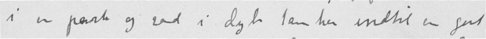i en park og sad i dybe tanker indtil en gut
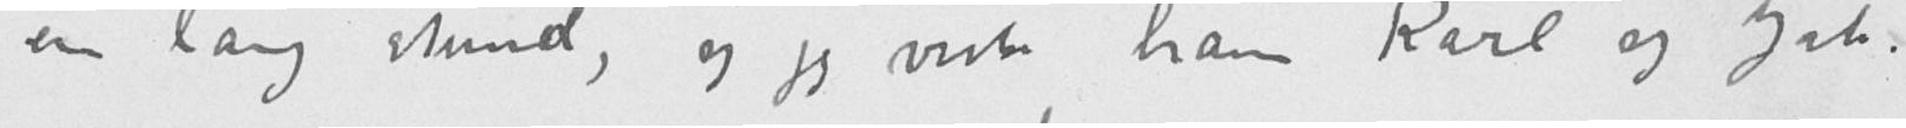en lang stund, og jeg viste ham Karl og Jak.
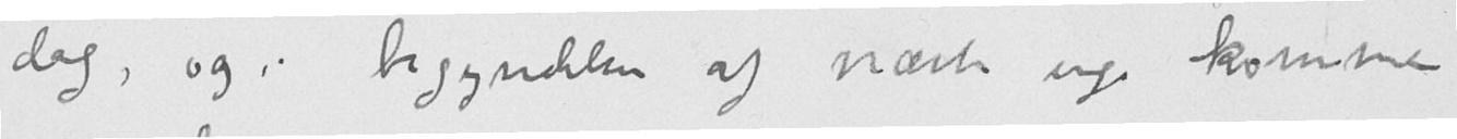dag, og i begyndelsen af næste uge kommer
